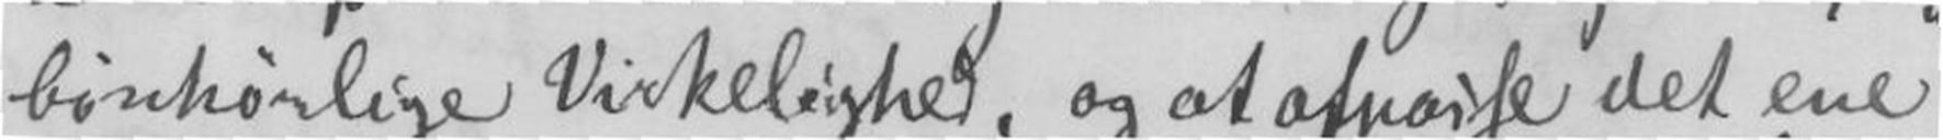bønhørlige Virkelighed, og at afpasse det ene
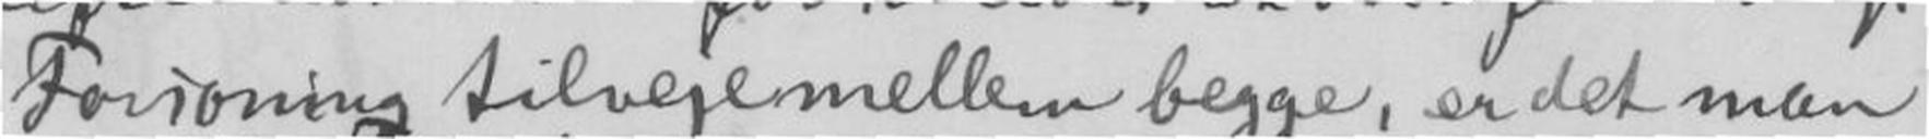Forsoning tilveje mellem begge, er det man
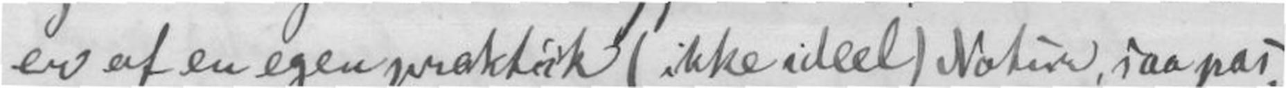er af en egen praktisk (ikke ideel) Natur, saa pas-
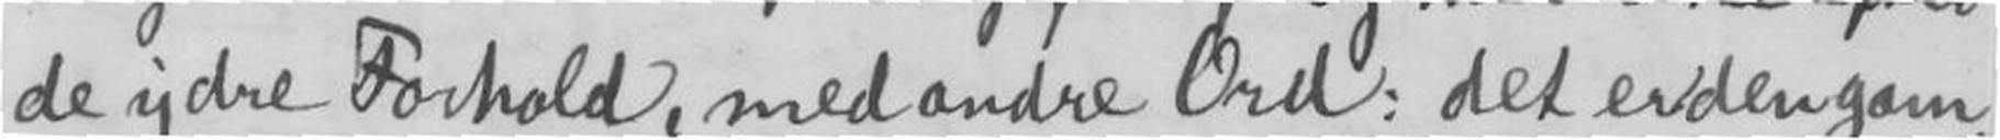de ydre Forhold, med andre Ord; det er en gam-
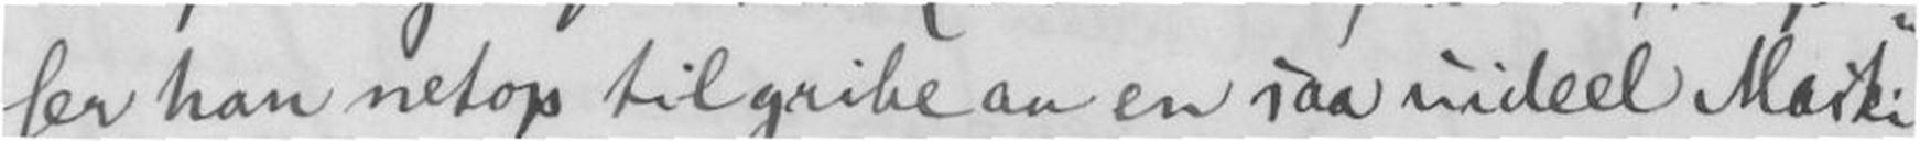ser han netop til gribe an en saa uideel Maski-
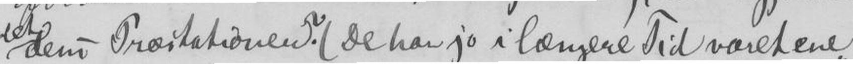dens Præstationer? (De har jo i længere Tid været ene
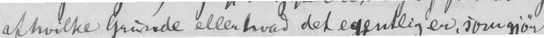af hvilke Grunde eller hvad det egentlig er, som gjør
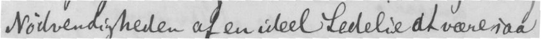Nødvendigheden af en ideel Ledelse at være saa
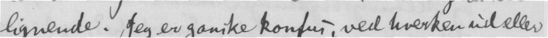lignende. Jeg er ganske konfus, ved hverken ud eller
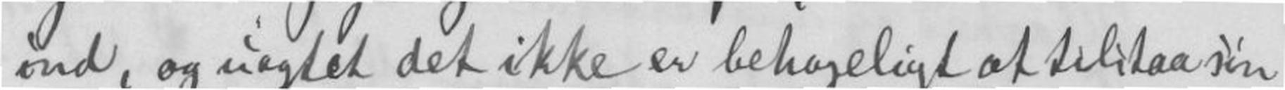ind, og uagtet det ikke er behageligt at tilstaa sin
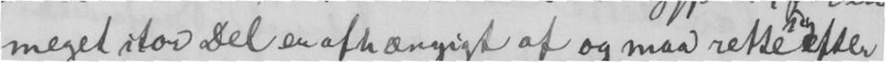meget stor Del en afhængigt af og maa rette sig efter
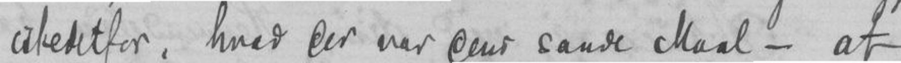-, hvad der var den sande Maal- af
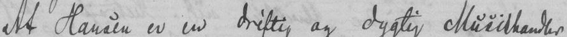At Hansen er en driftig og dygtig Musikhanler,
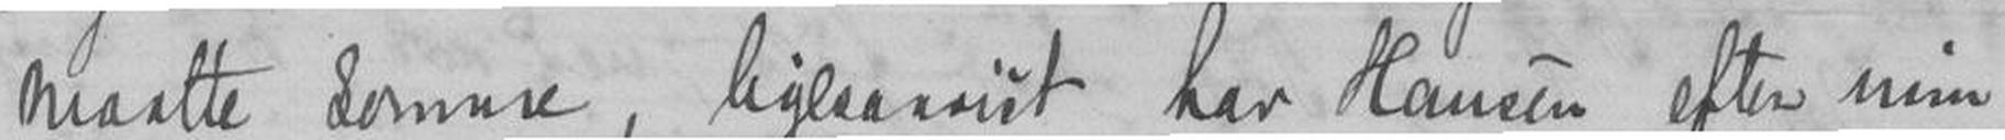maatte komme, har Hansen efter min
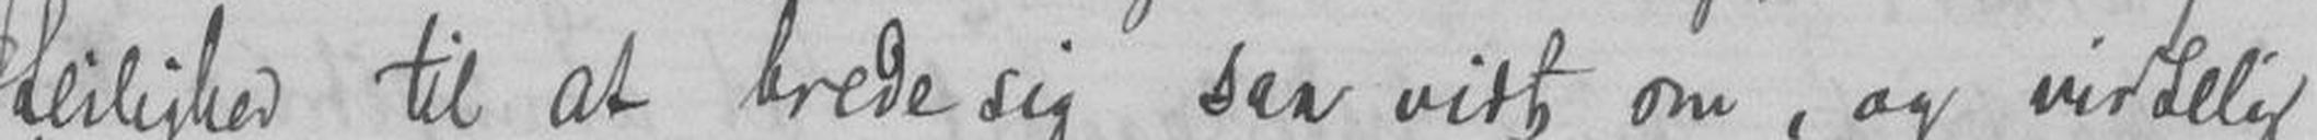leilighed til at brede sig saa vidt om, og virkelig
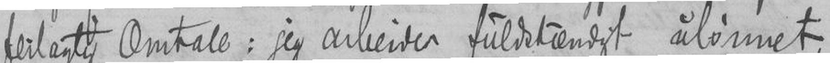feilagtig Omtale; jeg arbeider fuldstændigt ulønnet,
